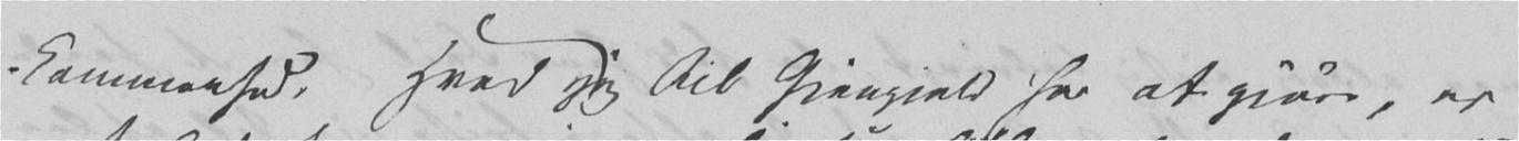kommenhed. Hvad jeg til Gjengjeld har at gjøre, er
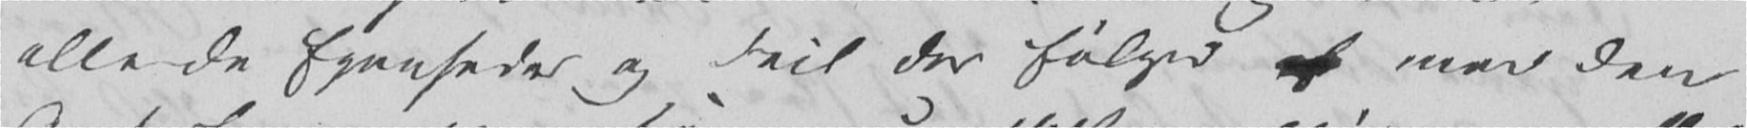alle de Egenheder og Feil der følger af med den
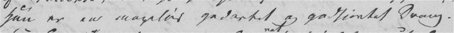Han er en mageløs godartet og godhjertet Dreng.
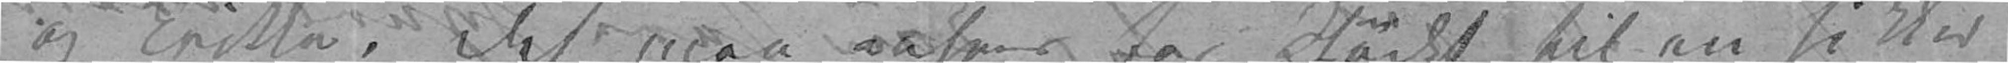og Erikka. Det maa ansees for Stødet til en sikker
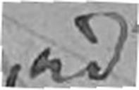ud
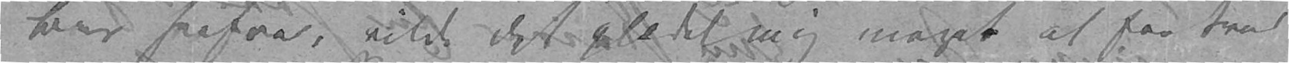Brev herfra, vilde det glædet mig meget at faa Svar
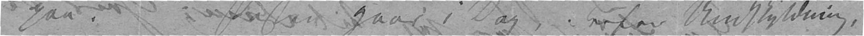paa. Posten gaar i Dag, derfor Undskyldning,
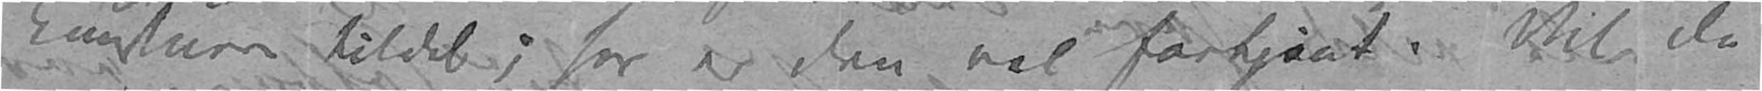Kunstnere tildel; her er den vel fortjent. Vil De
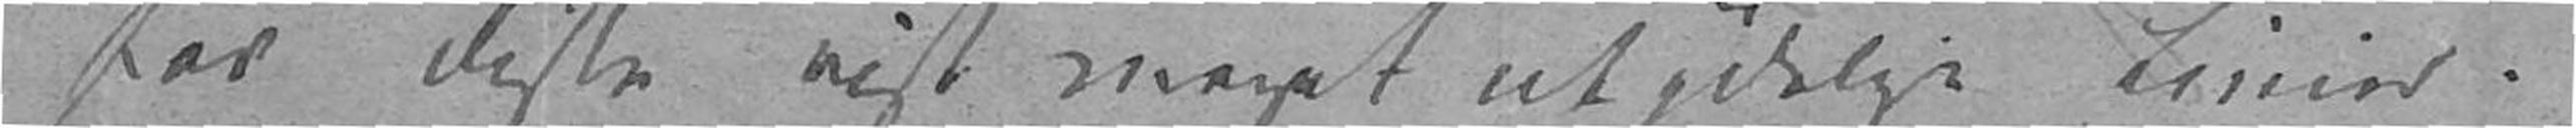for disse vist meget utydelige Linier.
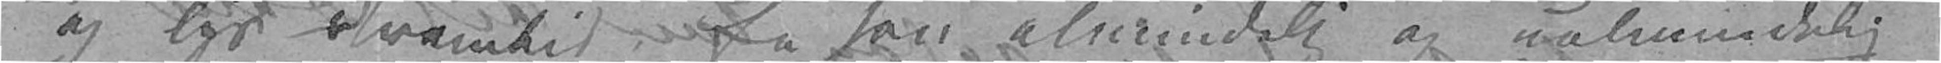og lys Fremtid. En saa almindelig og ualmindelig
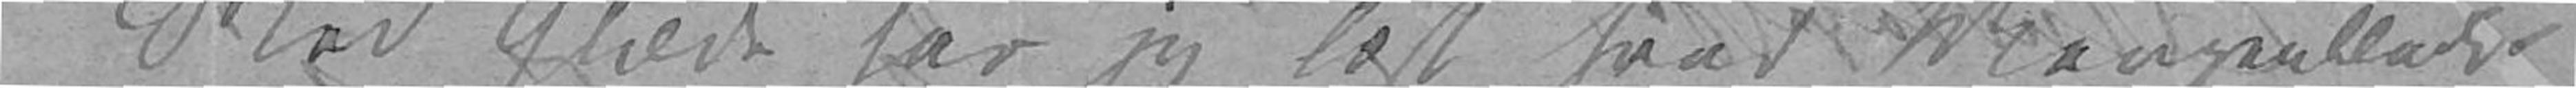Med Glæde har jeg læst hvad Morgenbladet
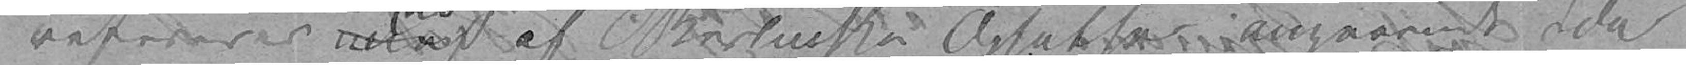refererer udaf af Berlinske Opsatser angaaende Ida
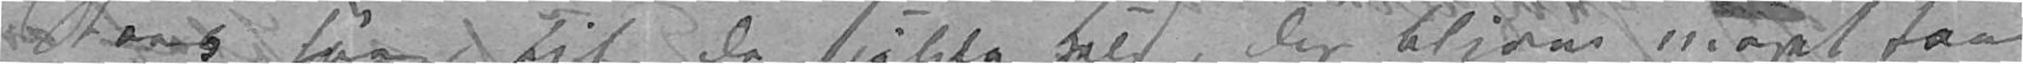Roes hører til de sjeld[n]e Held, der bliver meget faa
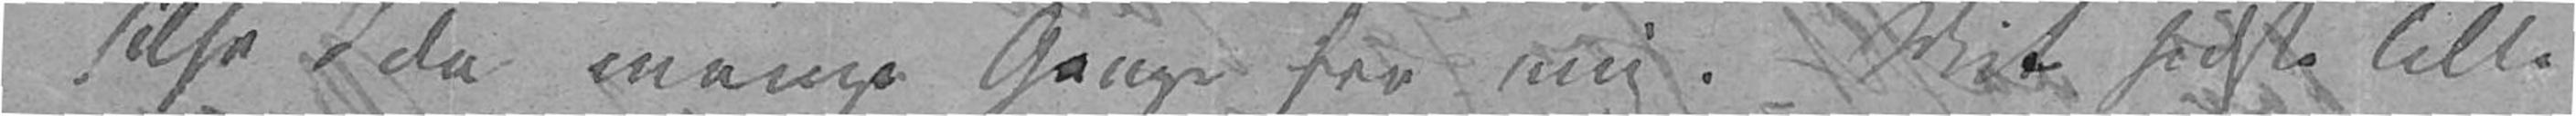hilse Ida mange Gange fra mig. Mit sidste lille
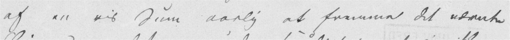af en vis Sum aarlig at fremme det nævnte
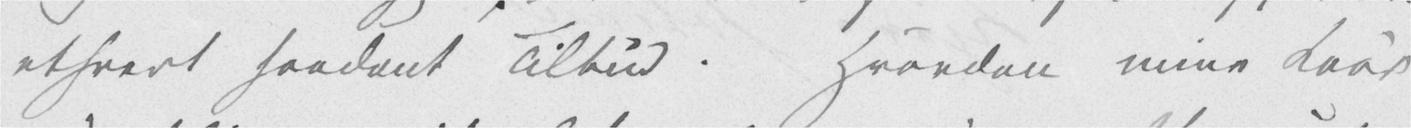ethvert saadant Tilbud. Hvordan mine Kaar
